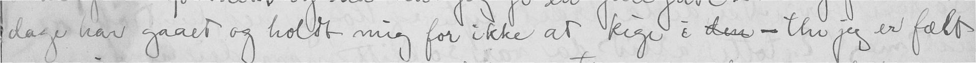dage har gaaet og holdt mig for ikke at kige i  - thi jeg er fælt
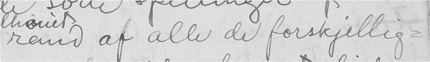rund af alle de forskjellig-
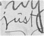just
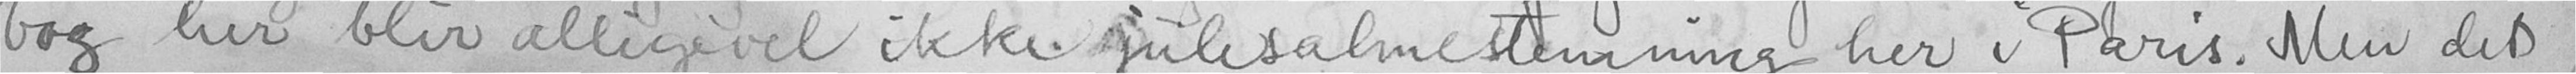bog her blir allegevel ikke julesalmestemning her i Paris. Men det
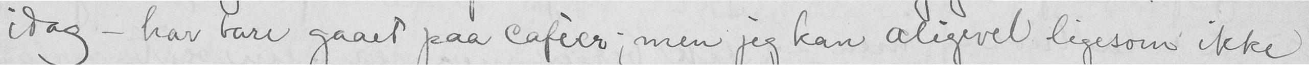idag - har bare gaaet paa caféer; men jeg kan aligevel ligesom ikke
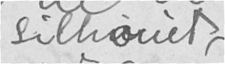silhouet
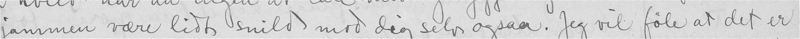jammen være lidt snild mod dig selv ogsaa. Jeg vil føle at det er
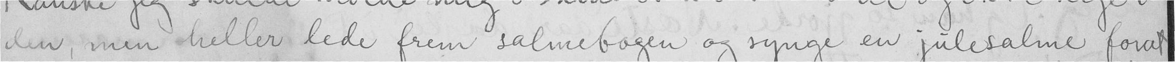den, men heller lede frem salmebogen og synge en julesalme forat
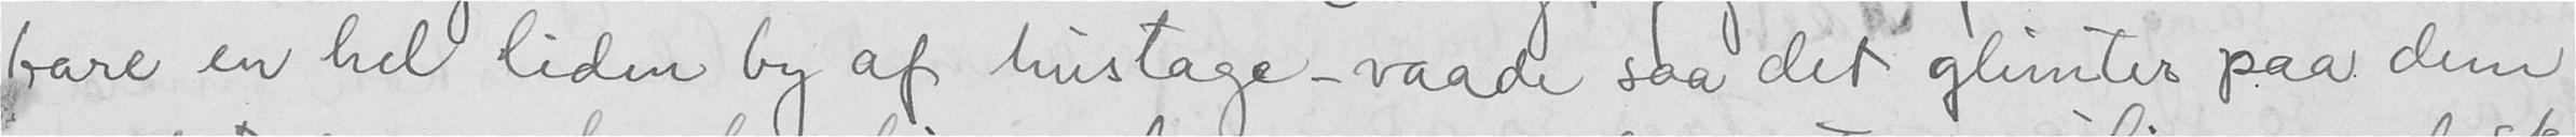bare en hel liden by af hustage - vaade saa det glimter paa dem
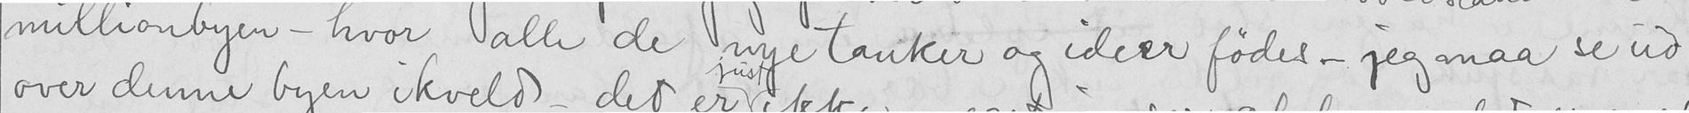millionbyen, - hvor alle de nye tanker og ideer fødes - jeg maa se ud
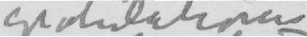gratulationer
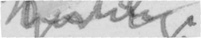Hjertelige
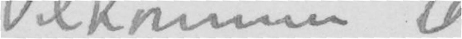Velkommen til
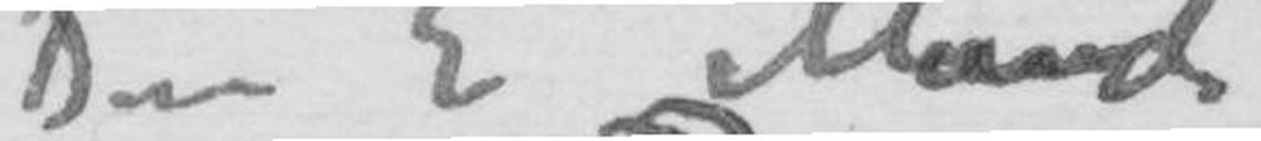Din E Munch
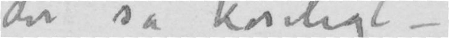det så koseligt –
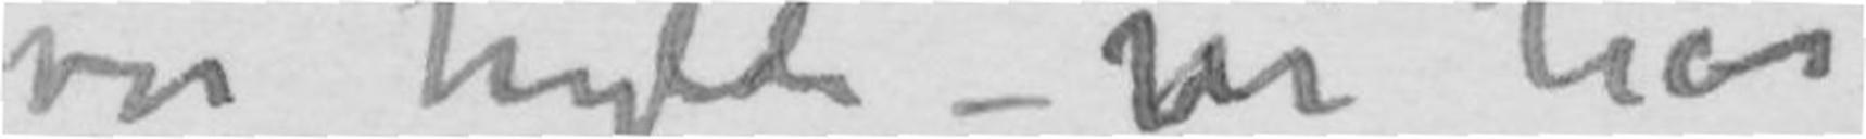vor hylde – vi har
